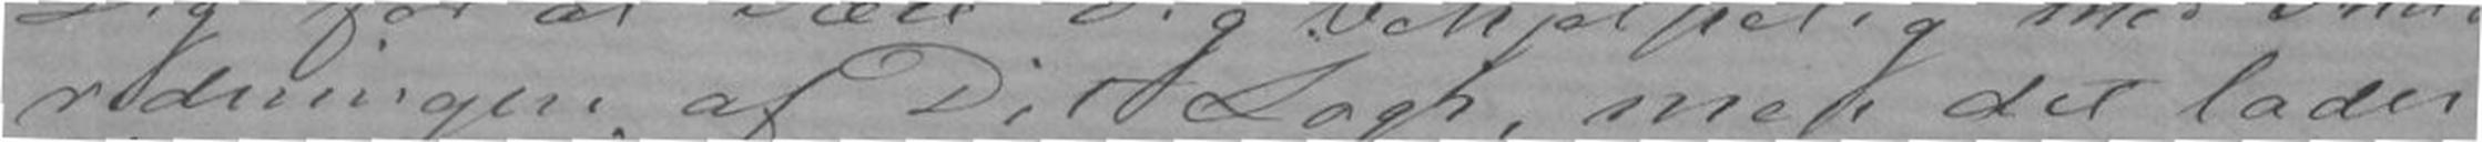redningen af Dit Logi, men det lader
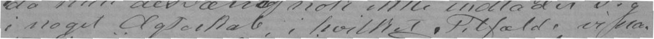i noget Ægteskab, i hvilket Tilfælde vi na-
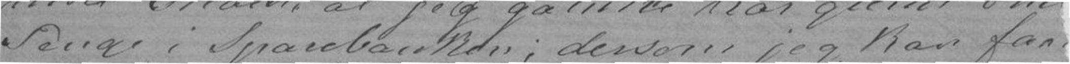Penge i Sparebanken; dersom jeg kan faa
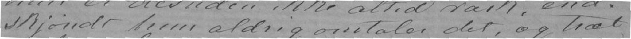skjøndt hun aldrig omtaler det, og træt
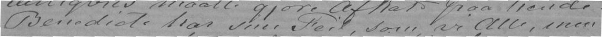Benedicte har sine Feil, som vi Alle, men
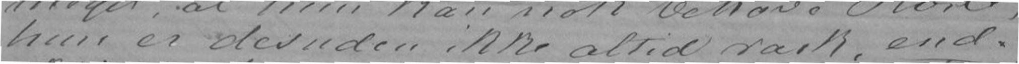hun er desuden ikke altid rask, end-
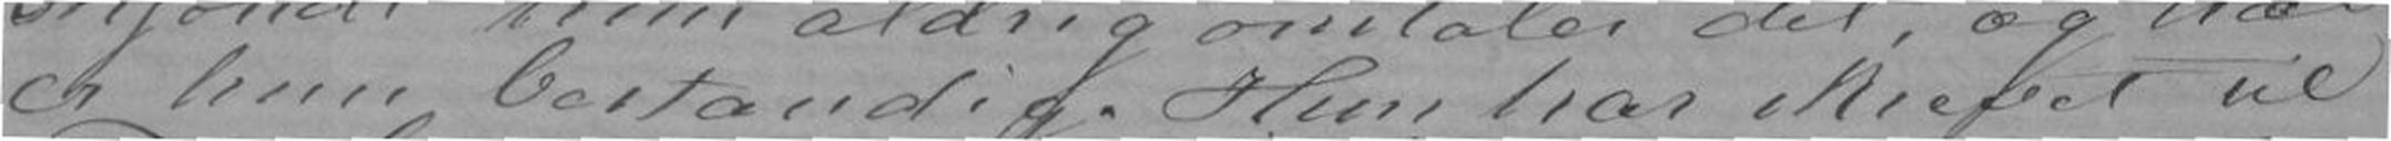er hun bestandig. Hun har skrevet til
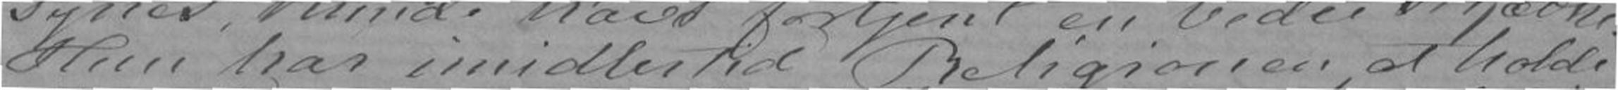Hun har imidlertid Religionen at holde
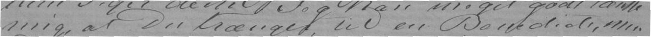mig at Du trænger til en Benedicte, men
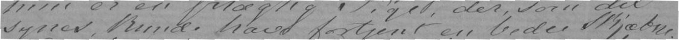synes kunde have fortjent en bedre Skjæbne.
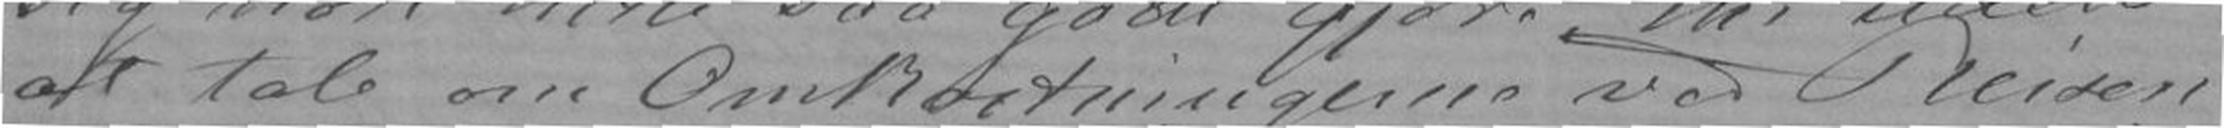at tale om Omkostningerne ved Reisen
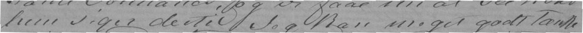hun siger dertil. Jeg kan meget godt tænke
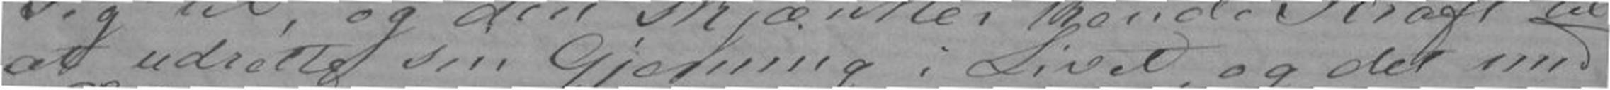at udrette sin Gjerning i Livet, og det med
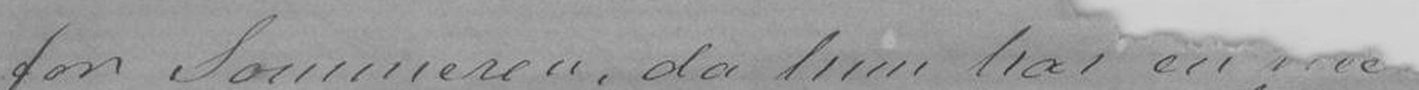for Sommeren, da hun har en
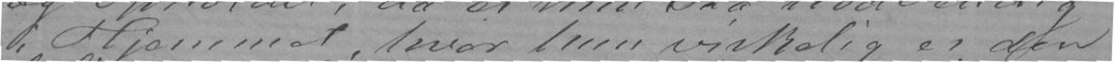i Hjemmet, hvor hun virkelig er den
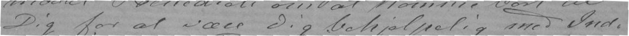Dig for at være Dig behjelpelig med Ind-
